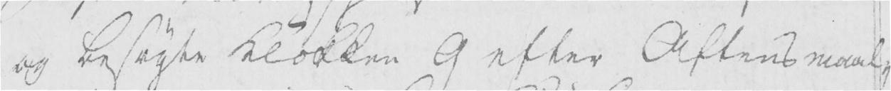og besøgte Klokken 9 efter Aftensmad
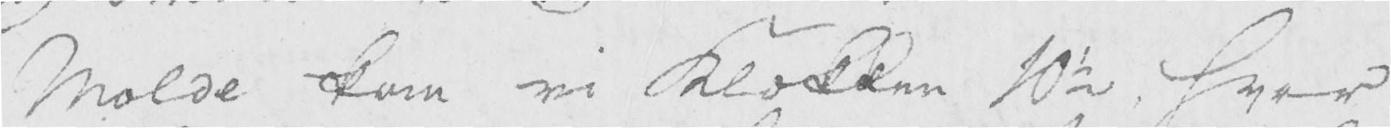Molde kom vi Klokken 10 1/2, hvor
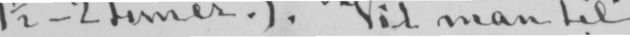(1½-2 timer.). Vil man til
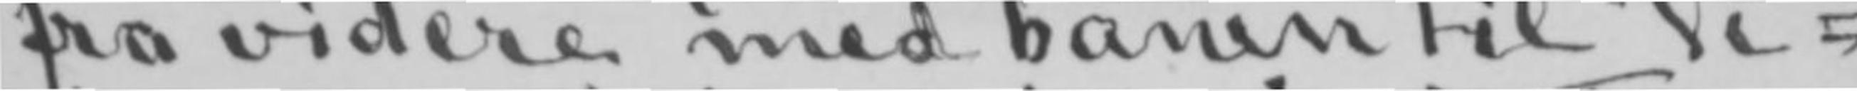fra videre med banen til Vi-
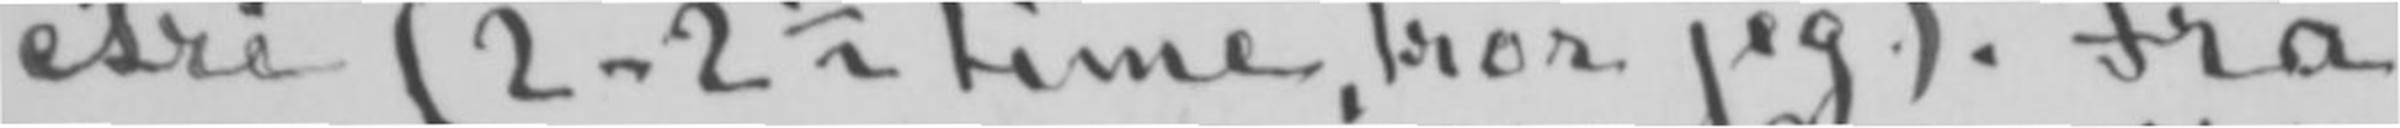etri (2-2½ time, tror jeg.). Fra
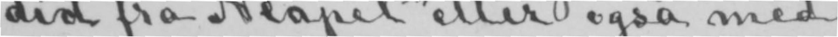did fra Neapel eller også med
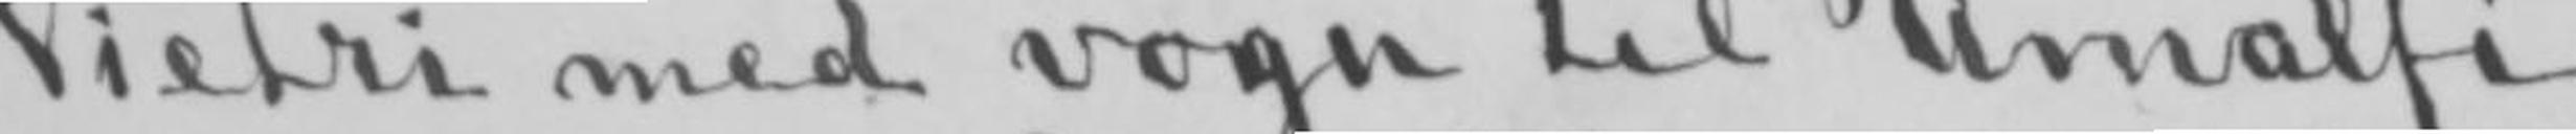Vietri med vogn til Amalfi,
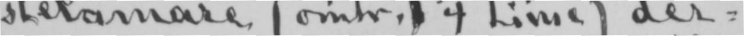stelamare (omtr. 1¼ time) der-
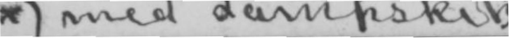x med dampskib.
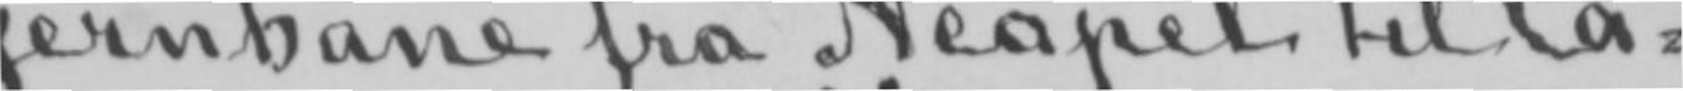Jernbane fra Neapel til Ca-
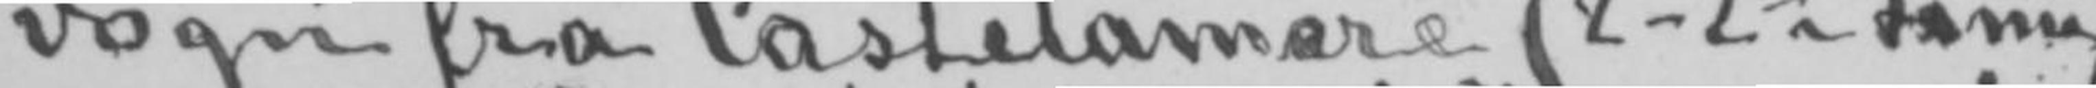vogn fra Castelamare (2-2½ time)
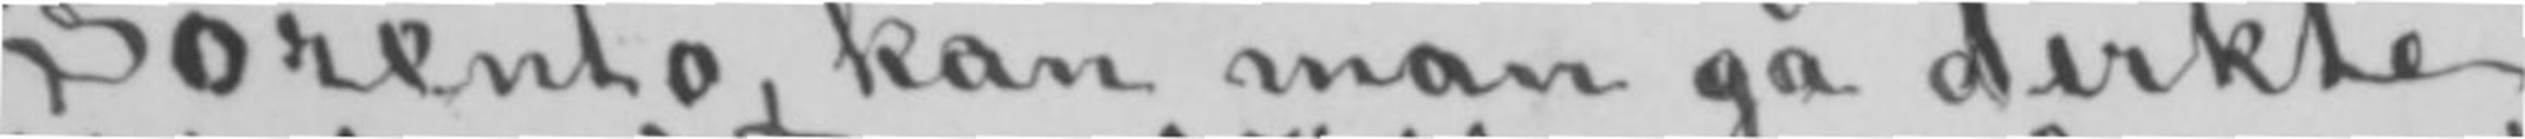Sorento, kan man gå dirkte
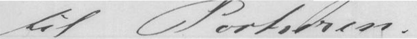til Portieren.
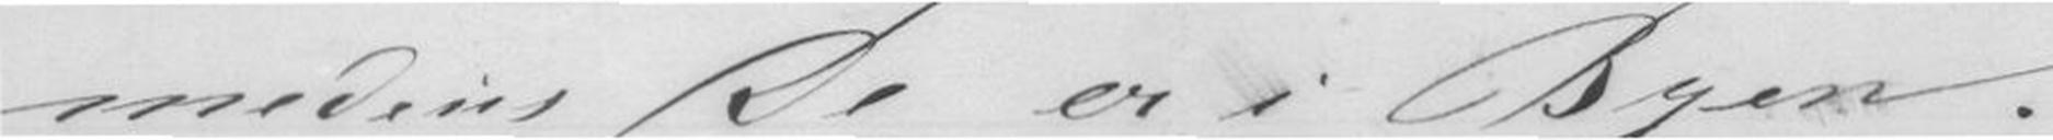medens De er i Byen.
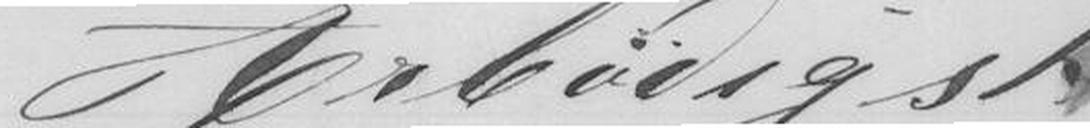Ærbødigste
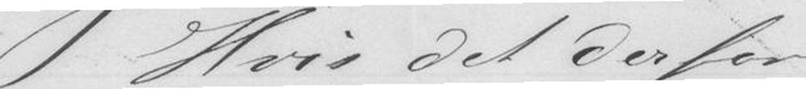Hvis det derfor
